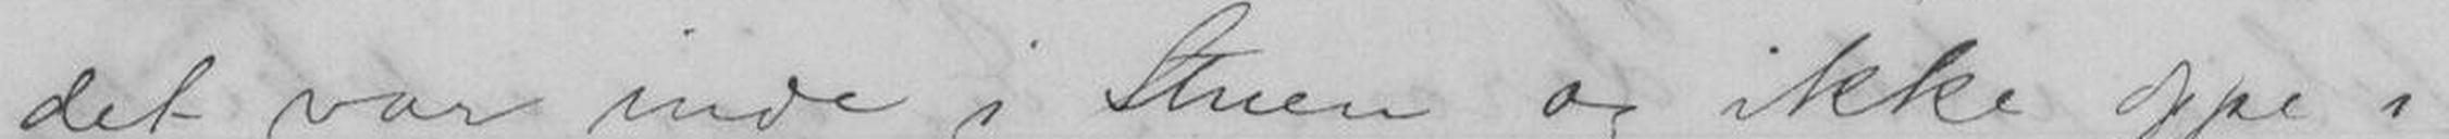det var inde i Stuen og ikke oppe i
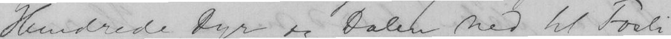Hundrede Dyr og Dalen ned til Fosli
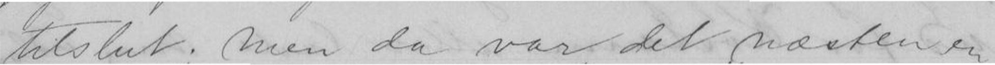tilslut; men da var det næsten en
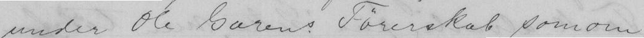under Ole Garens Førerskab, som om
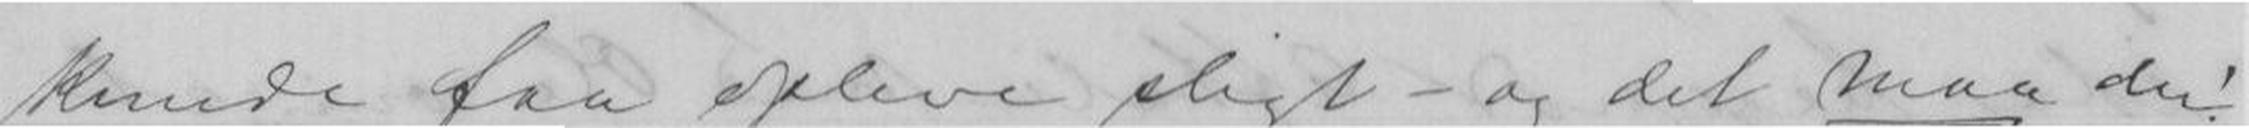kunde faa opleve sligt - og det maa du!
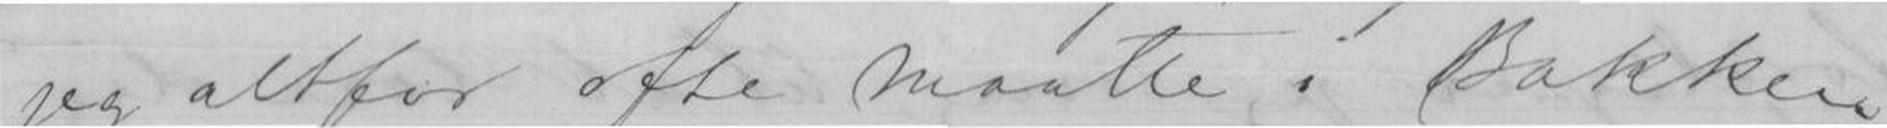jeg altfor ofte maatte i Bakken
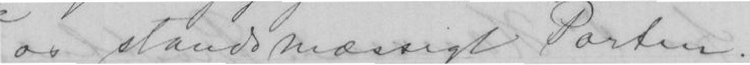os standsmæssigt Porten!
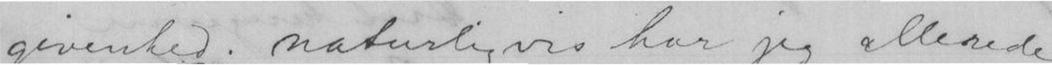givenhed; naturligvis har jeg allerede
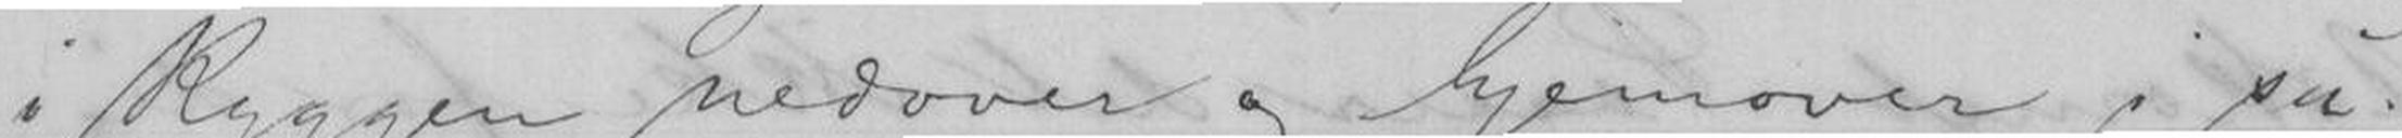i Ryggen nedover og hjemover i su-
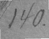140.
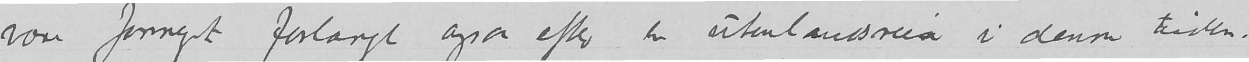være formeget forlangt ogsaa efter en utenlandsreise i denne tiden.
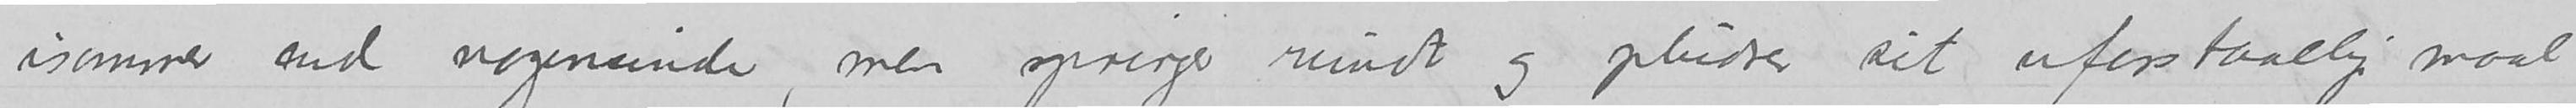isommer end nogensinde, men springer rundt og pludrer sit uforstaaelige maal
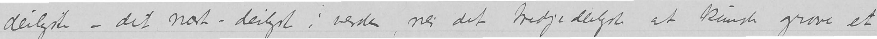deiligste – det næst-deiligste i verden, nei det tredje deiligste at
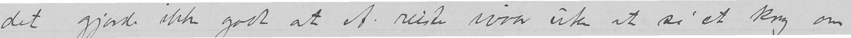det gjorde ikke godt at A. reiste ivaar uten at si et kny om
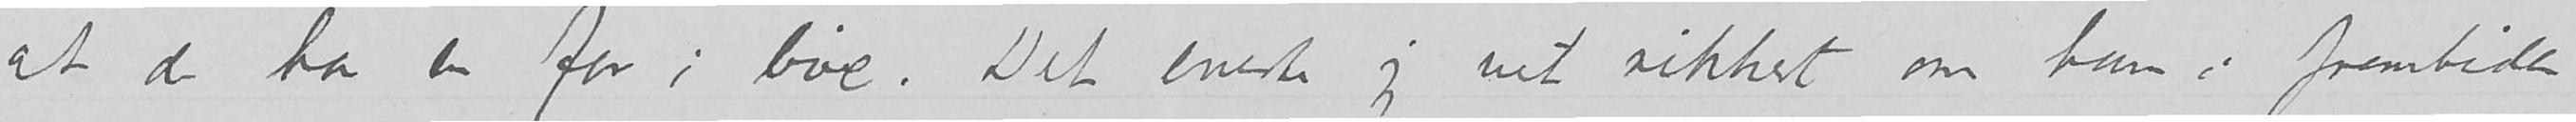at de har en far i live.  Det eneste jeg vet sikkert om ham i fremtiden
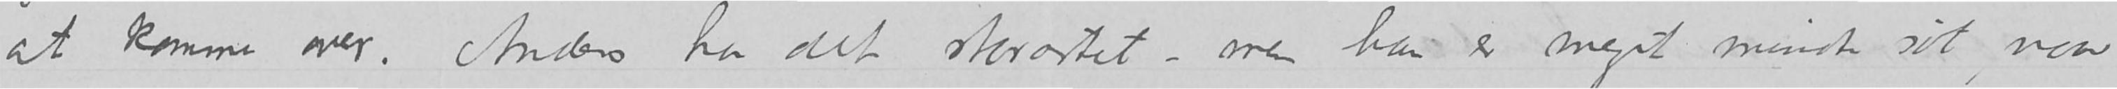at komme over.  Anders har det storartet – men han er meget mindre søt, naar
